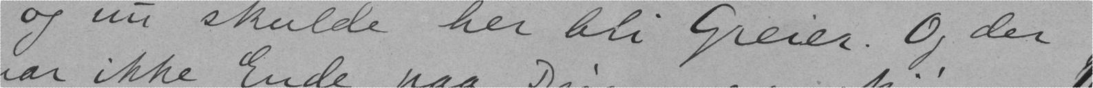og nu skulde her bli Greier. Og der
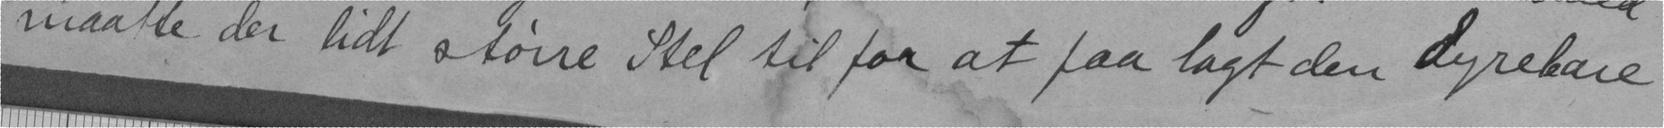maatte der lidt større Stel til for at faa lagt den Dyrebare
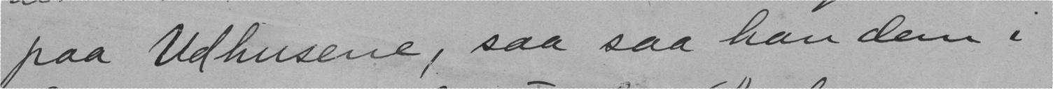paa Udhusene, saa saa han dem i
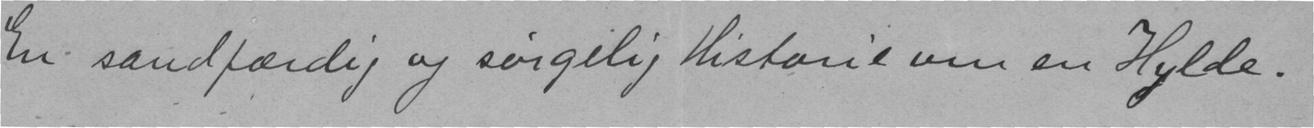En sandfærdig og sørgelig Historie om en Hylde.
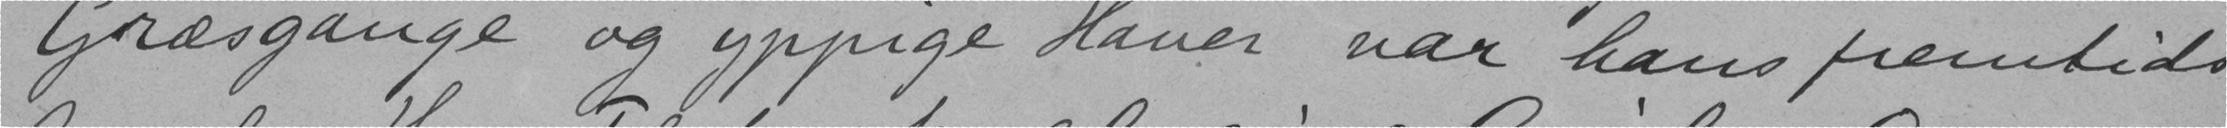Græsgange og yppige Haver var hans fremtids
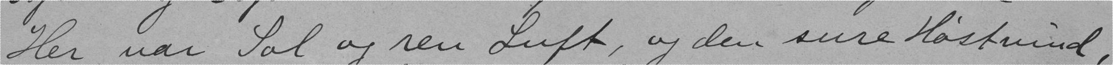Her var Sol og ren Luft, og den sure Høstvind,
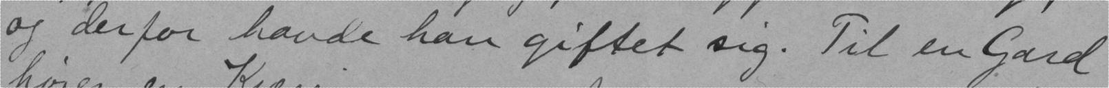og derfor havde han giftet sig. Til en Gard
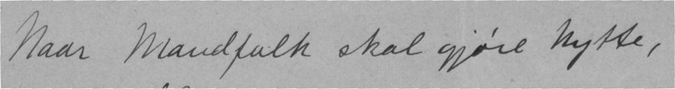Naar Mandfolk skal gjøre nytte,
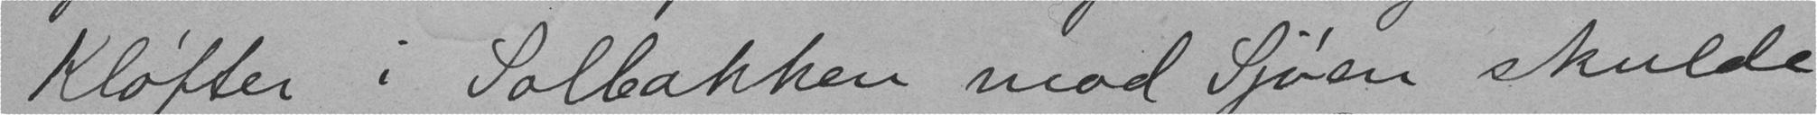Kløfter i Solbakken mod Sjøen skulde
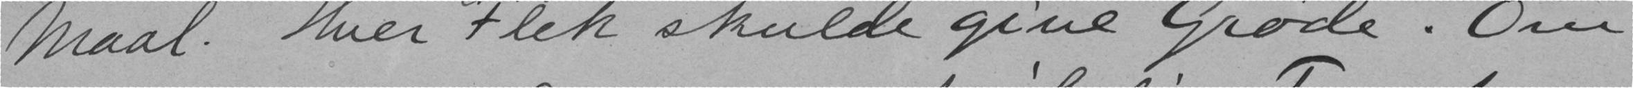Maal. Hver Flek skulde give Grøde. Om
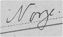Norge.
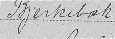Bjerkebæk
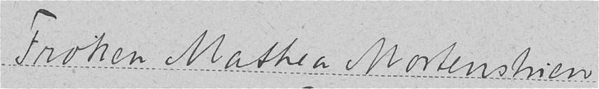Frøken Mathea Mortenstuen
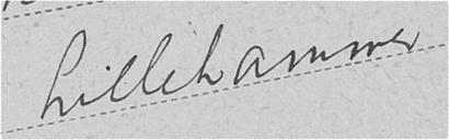Lillehammer
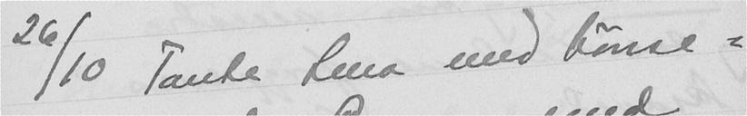26/10 Tante Lena med hønse-
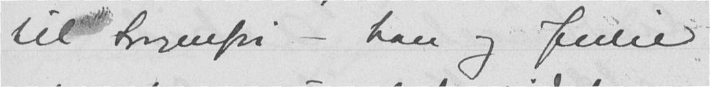til Sorgenfri - han og Julie
